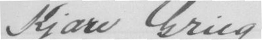Kjære Grieg!
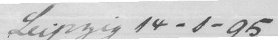Leipzig 14-1-95
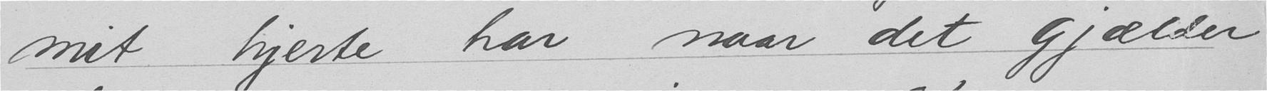mit hjerte har naar det gjælder
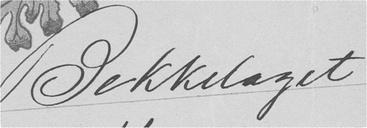Bekkelaget
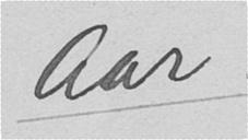aar.
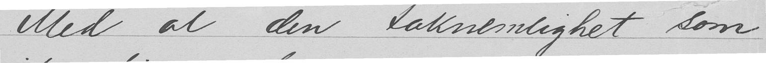Med al den taknemlighet som
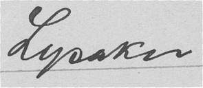Lysaker
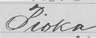Pioka
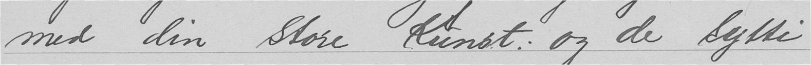med din Store Kunst, og de sytti
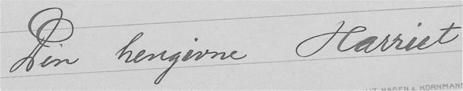Din hengivne Harriet
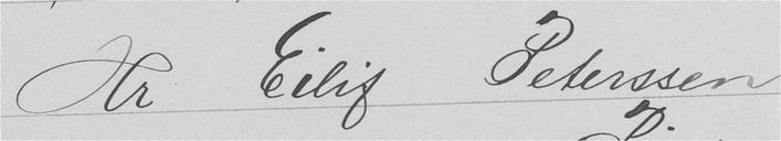Hr Eilif Peterssen
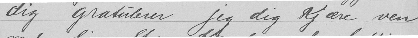dig gratulerer jeg dig Kjære ven
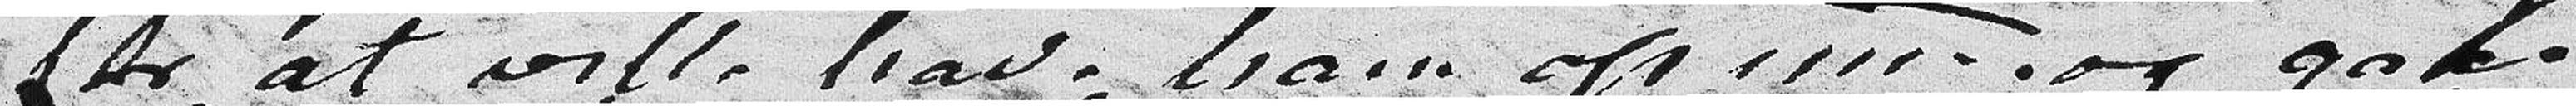for at ville have ham opmed og gaae
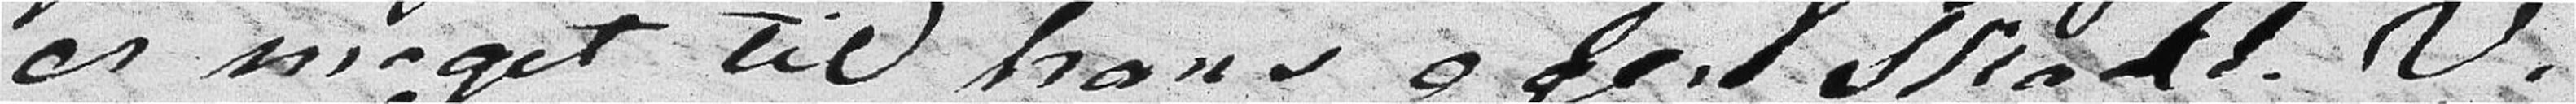er meget til hans egen Skade. Vi
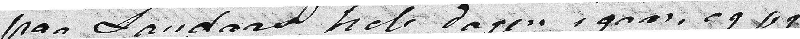paa LAndaas hele dagen igaar, og jeg
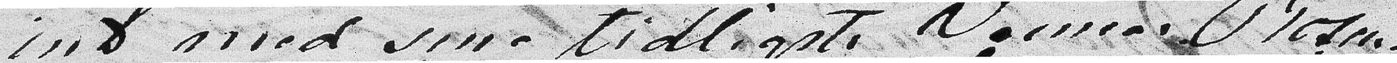ind med sine tidligste Venner, Rosen-
